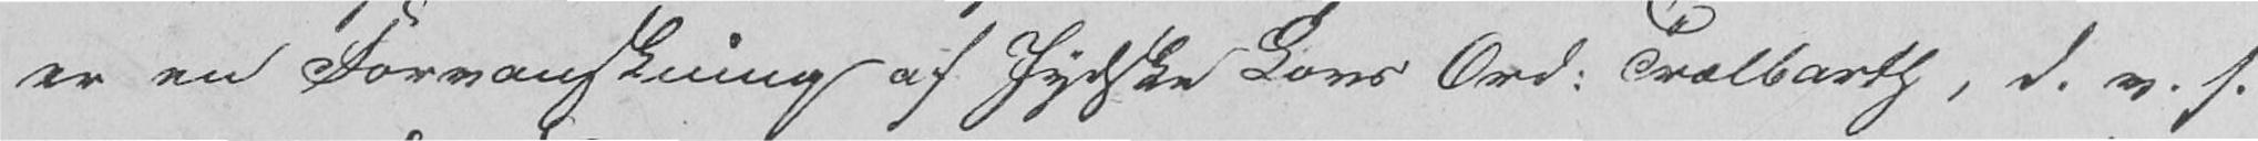er en Forvanskning af Jydske Lovs Ord: Trælbarth, d. v. s.
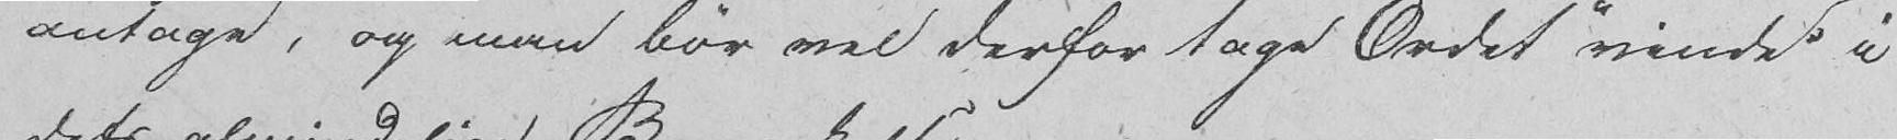antage, og man bør vel derfor tage Ordet "vinde" i
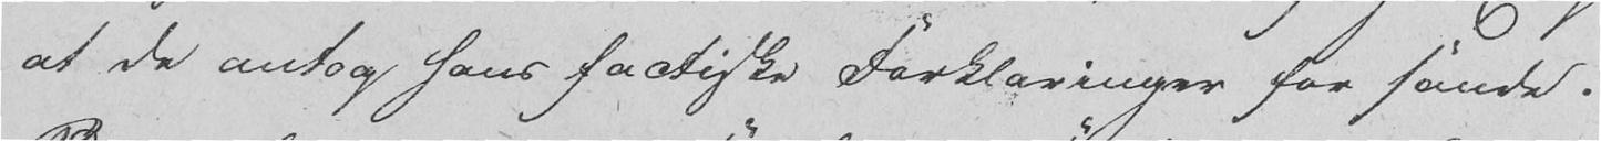at de antog hans factiske Forklaringer for sande.
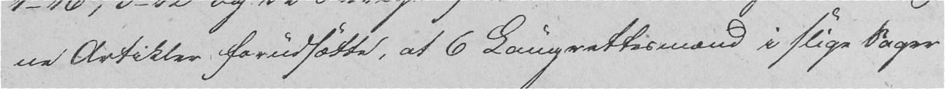ne Artikler forudsætte, at 6 Laugrettesmænd i slige Sager
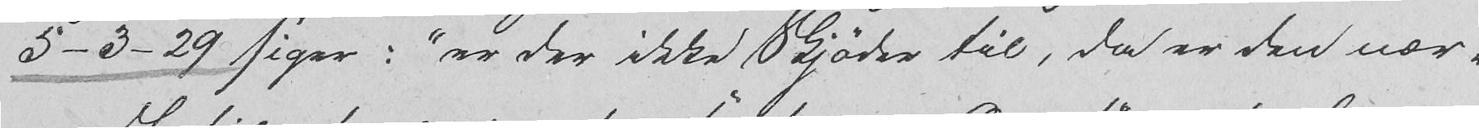5-3-29 siger: "er der ikke Skjøder til, da er den nær-
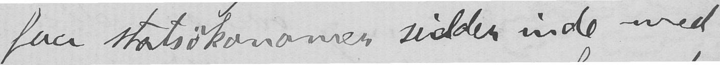faa statsøkonomer sidder inde med
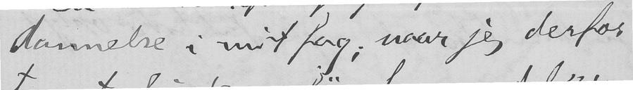dannelse i mit fag; naar jeg derfor
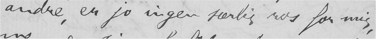andre, er jo ingen særlig ros for mig,
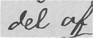del af
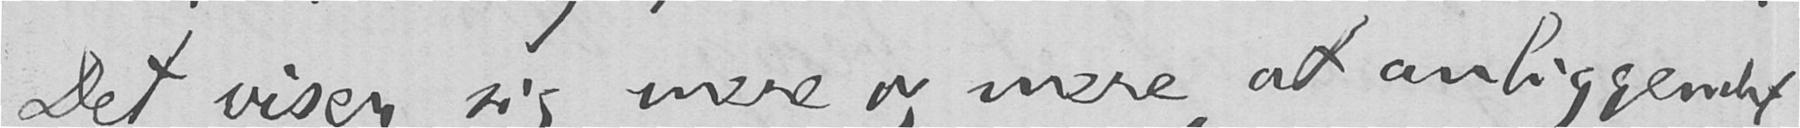Det viser sig mere og mere, at anliggendet
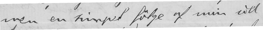men en simpel følge af min ud-
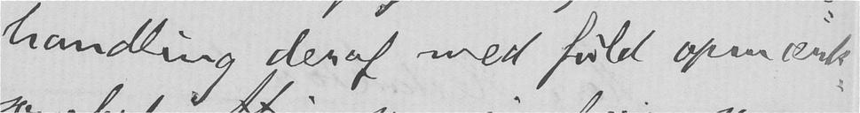handling deraf med fuld opmærk-
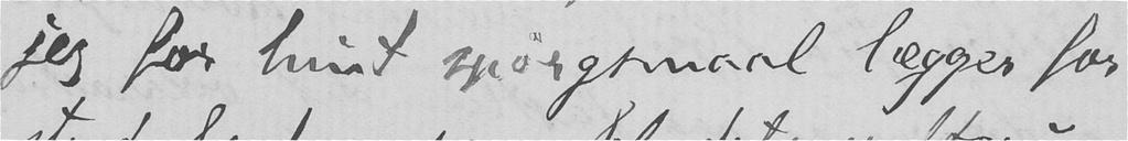jeg for hint spørgsmaal lægger for
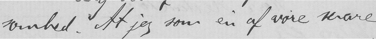somhed. At jeg som en af vore saare
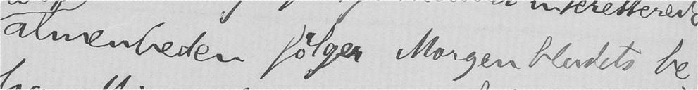almenheden følger Morgenbladets be-
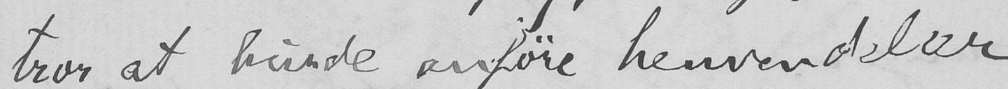tror at burde anføre henvendelser
